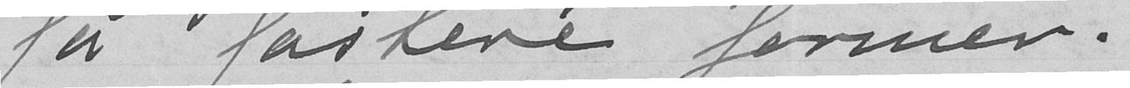få fastere former.
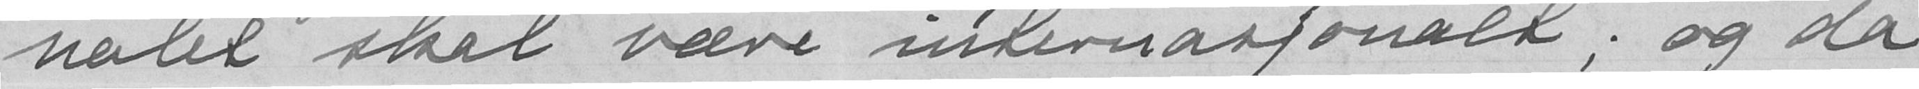nalet skal være internasjonalt; og da
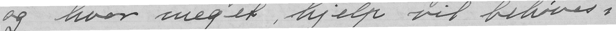og hvor meget, hjelp vil behøves i
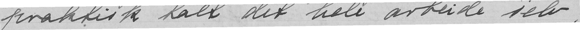praktisk talt det hele arbeide selv;
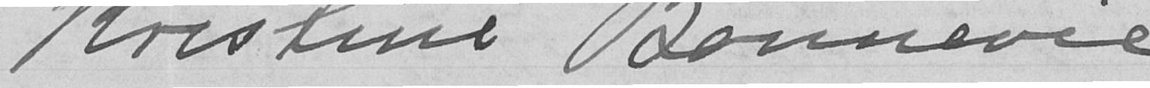Kristine Bonnevie
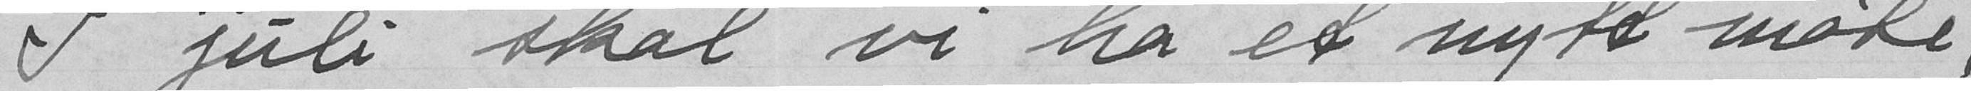I juli skal vi ha et nytt møte,
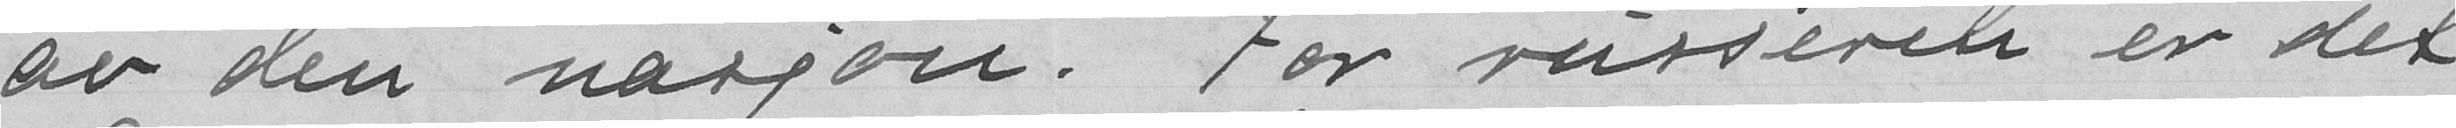av den nasjon. For russeren er det
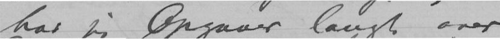har jeg Opgaver langt over
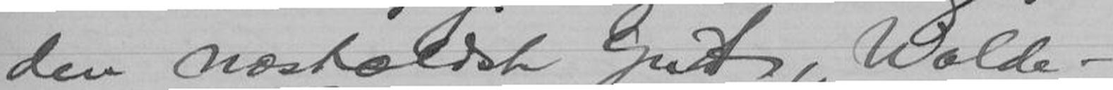den næstældste Gut, Walde-
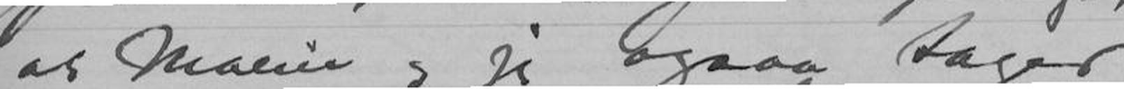at Marie og jeg ogsaa tager
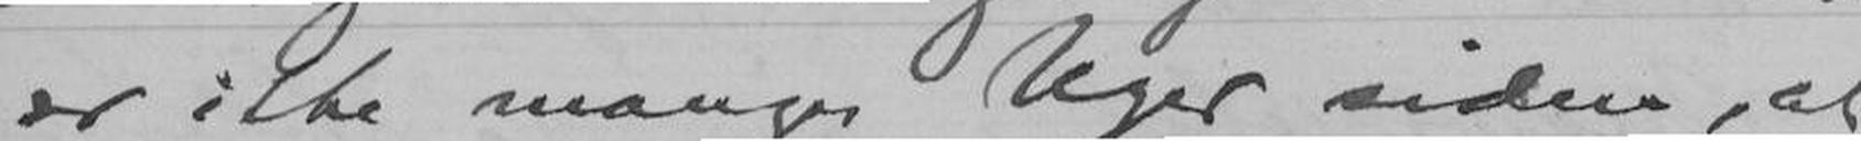er ikke mange Uger siden, at
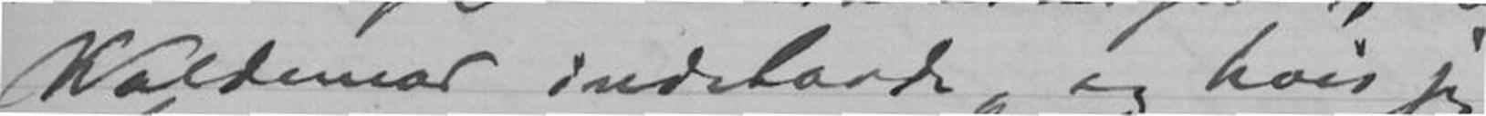Waldemar indehavde, og hvis jeg
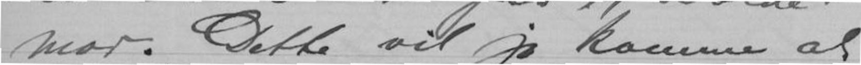mar. Dette vil jo komme at
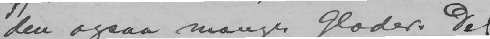den ogsaa mange Glæder. Det
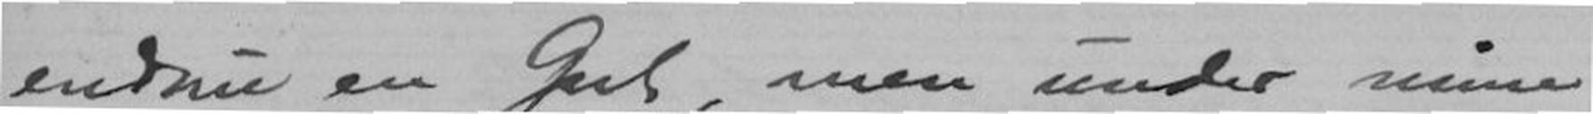endnu en Gut, men under mine
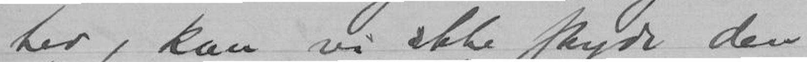her, kan vi ikke skyde den
